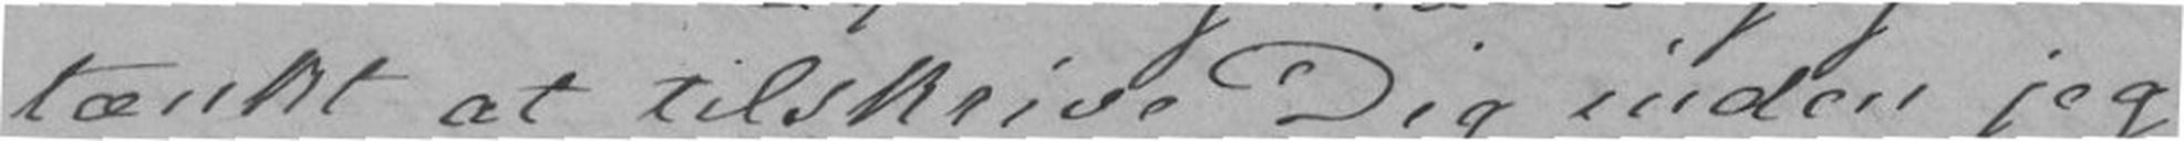tænkt at tilskrive Dig inden jeg
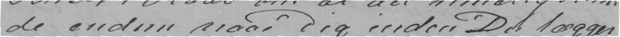de endnu naae Dig inden Du lægger
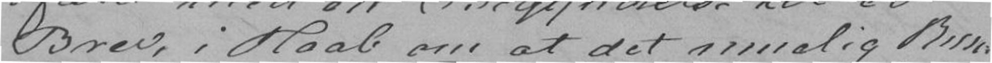Brev, i Haab om at det muelig kun-
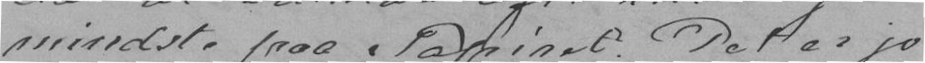mindste paa Papiret. Det er jo
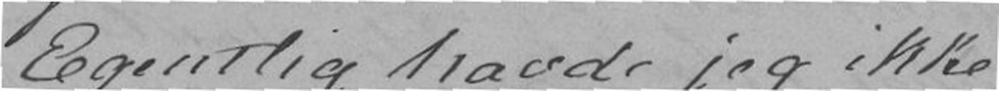Egentlig havde jeg ikke
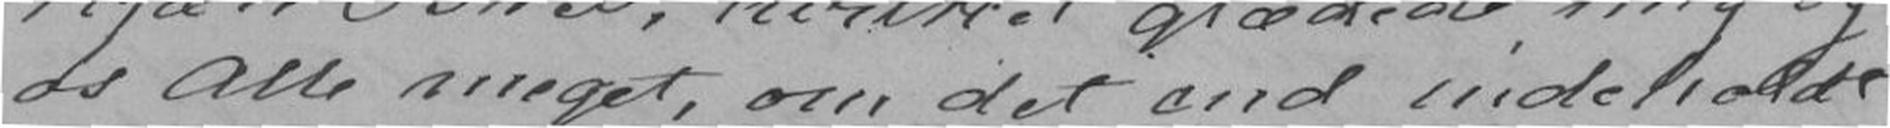os alle meget, om det end indeholdt
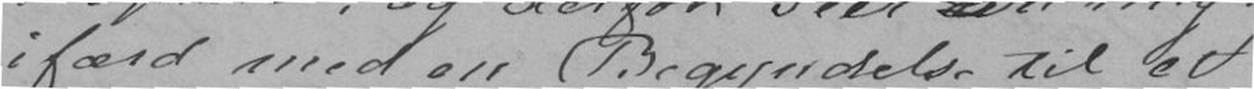ifærd med en Begyndelse til et
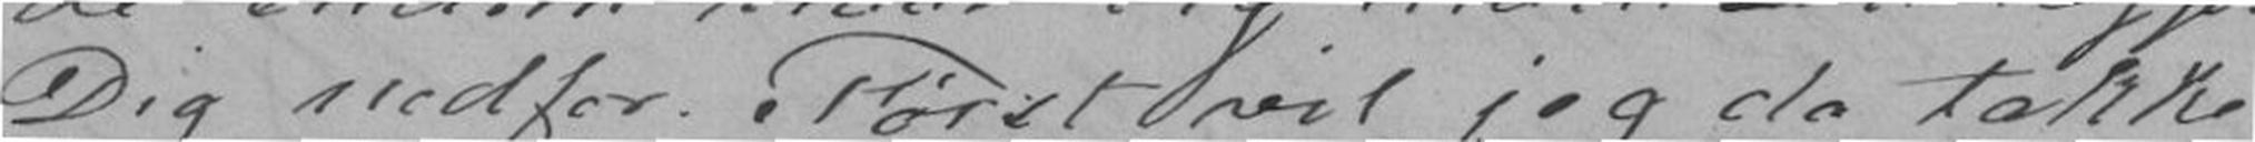Dig nedfor. Først vil jeg da takke
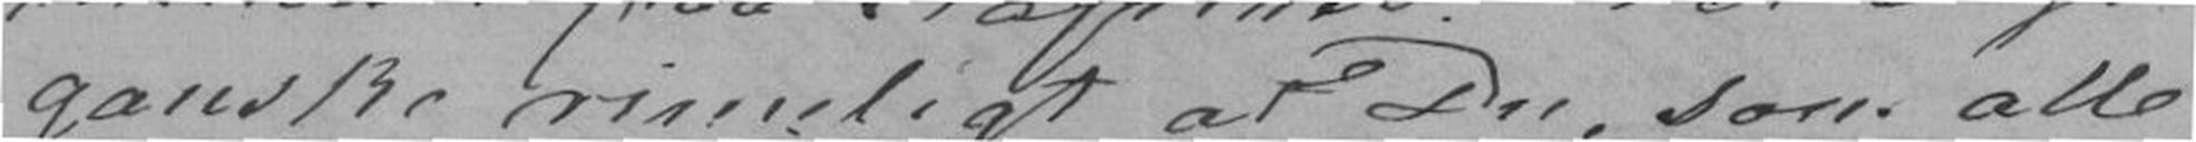ganske rimeligt at Du, som alle
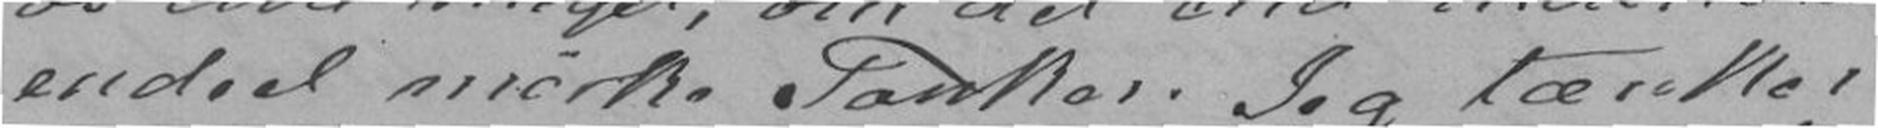endeel mørke Tanker. Jeg tænker
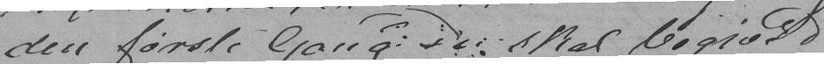den første Gang Du skal begive Dig
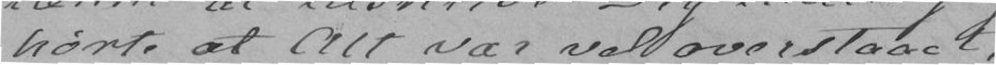hørte at Alt var vel overstaaet,
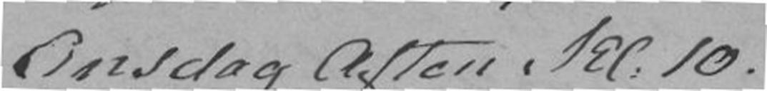Onsdag Aften Kl. 10.
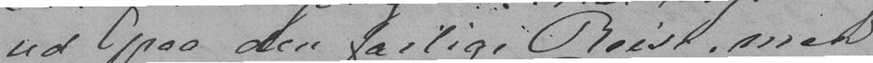ud paa den farlige Reise, men
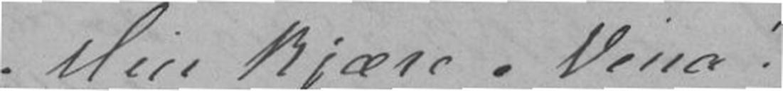Min kjære Nina!
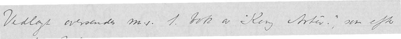Vedlagt oversendes ms. 1. bok av "Kong Artur.", som efter
